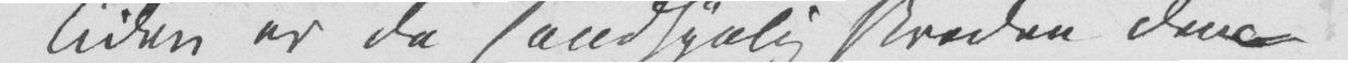Tiden er da sandsynlig skreden den
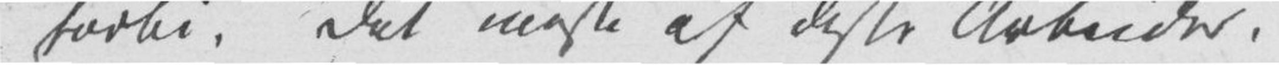forbi. Det meste af disse Arbeider,
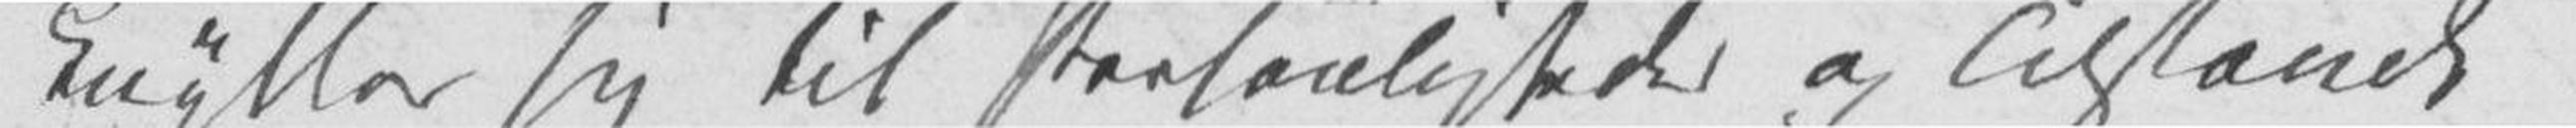knytter sig til Personligheder og Tilstande
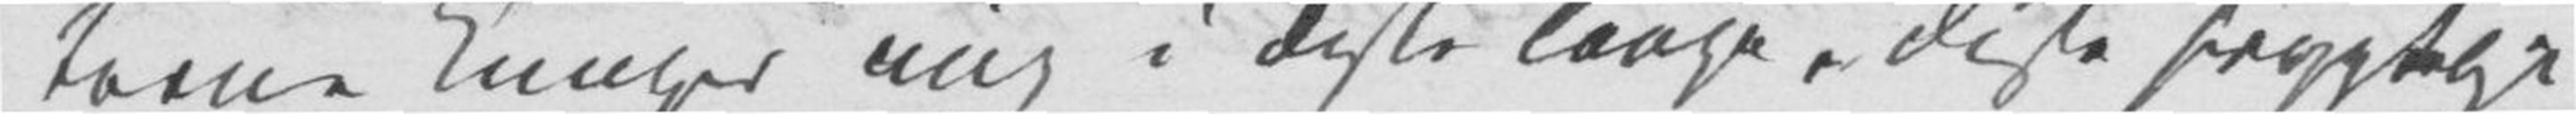terne knuger mig i disse lange, disse frygtelige
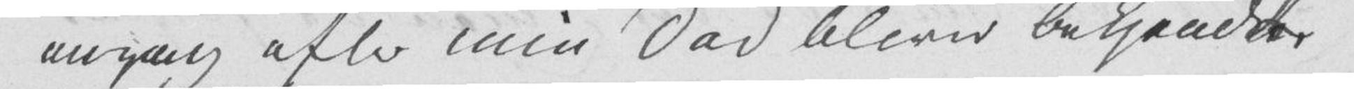engang efter min Død bliver bekjendt.
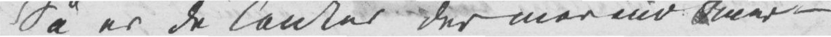Så er de Tanker der mer end Smer¬
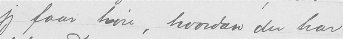jeg faar høre, hvordan du har
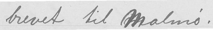brevet til Malmø.
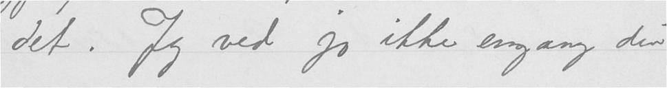det. Jeg ved jo ikke engang din
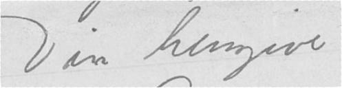Din hengivne
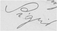Sigrid
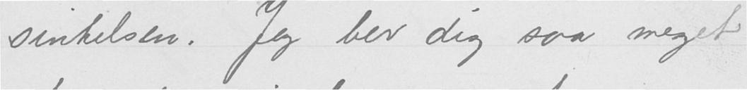sinkelsen. Jeg ber dig saa meget
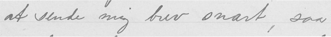at sende mig brev snart, saa
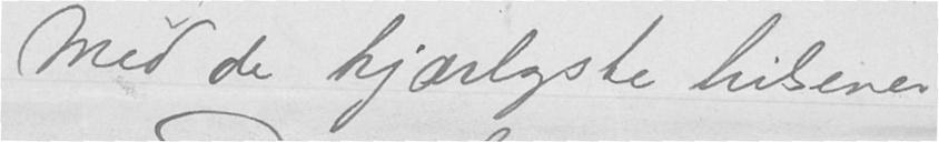Med de kjærligste hilsener
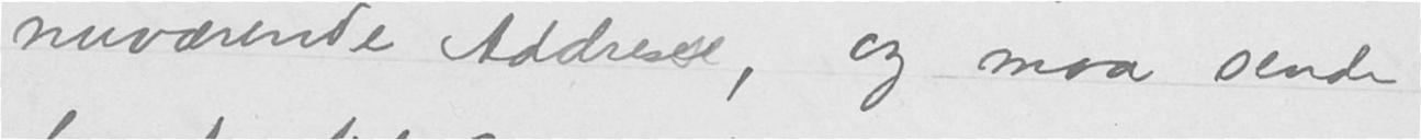nuværende Addresse, og maa sende
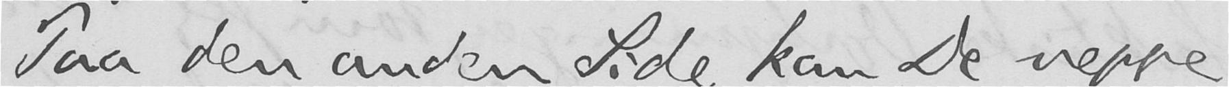Paa den anden Side kan De neppe
